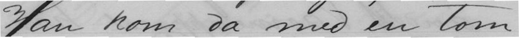Han kom da med en Tom
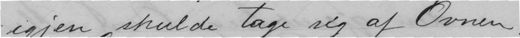igjen skulde tage sig af Ovnen.
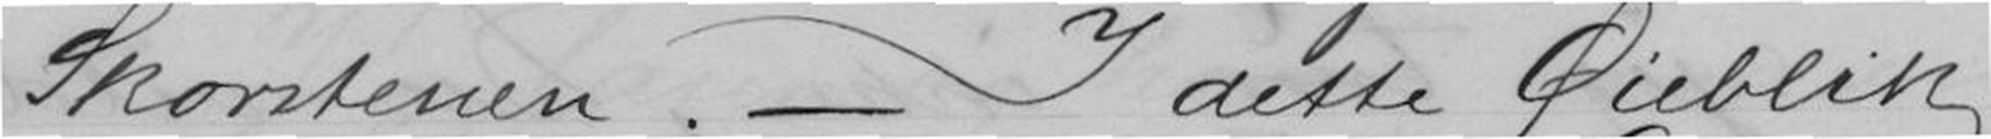Skorstenen. _ I dette Øieblik
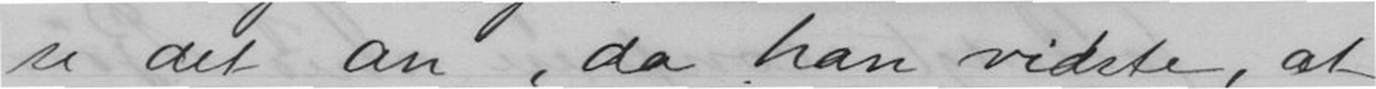se det an, da han vidste, at
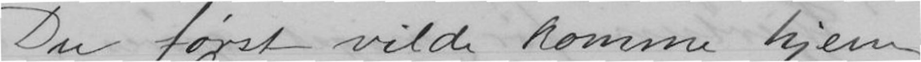Du først vilde komme hjem
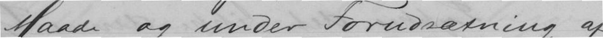Maade og under Forudsætningen af,
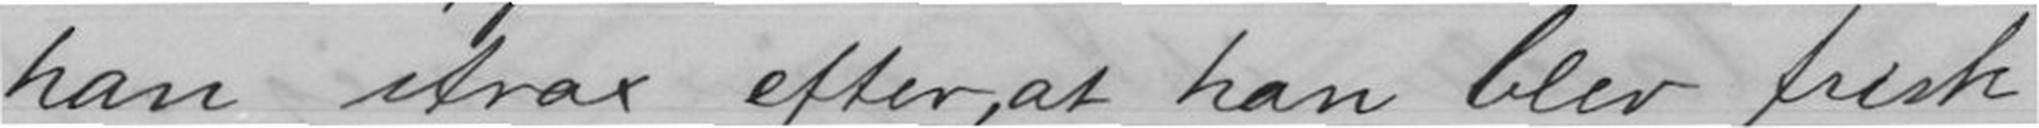han strax efterat han blev frisk
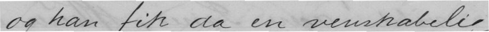og han fik da en venskabelig
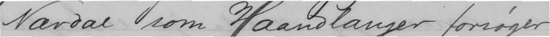Nævdal som Haandlanger forsøger
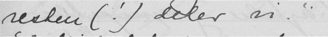resten (!) deler vi."
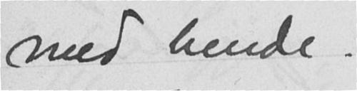med hende.
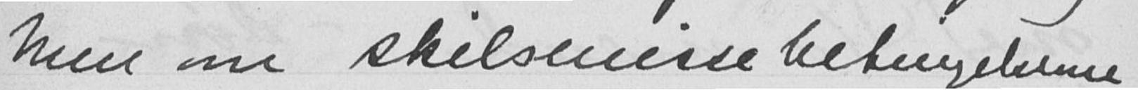Men om skilsmissebetingelserne
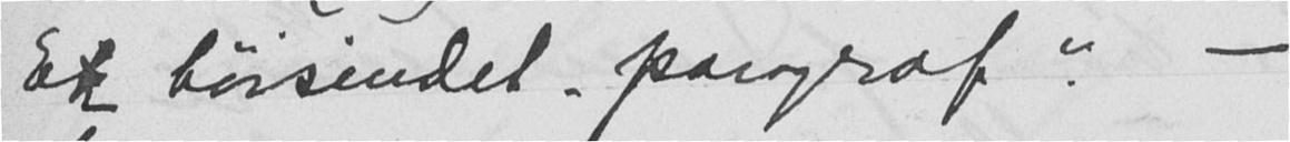En høisindet "paragraf". -
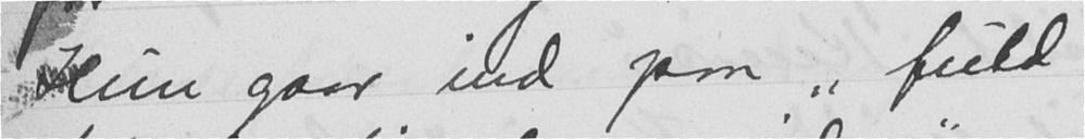Hun gaar ind paa "fuld
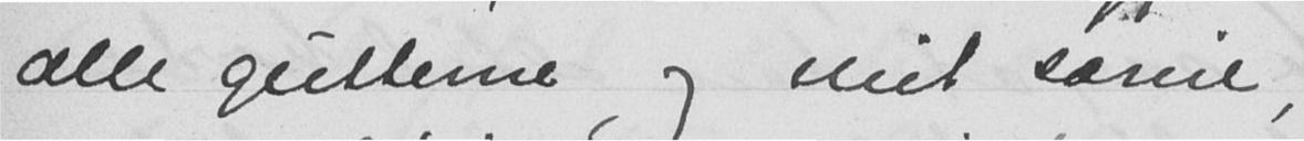alle gutterne og mit særeie,
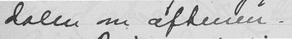dalen om aftenen.
